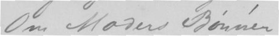Om Moders Bønner
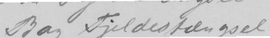Bag Fjeldestængsel,
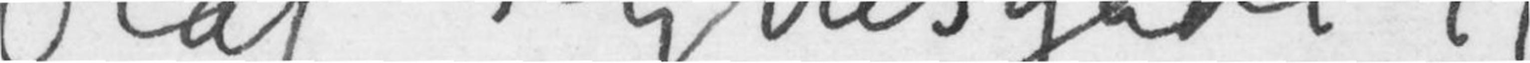Olaf Kyrres gade 11
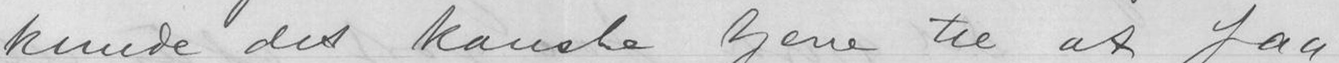kunde det kanske tjene til at faa
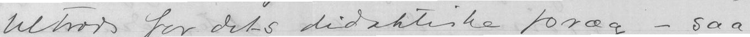tiltross for dets didaktiske præg - saa
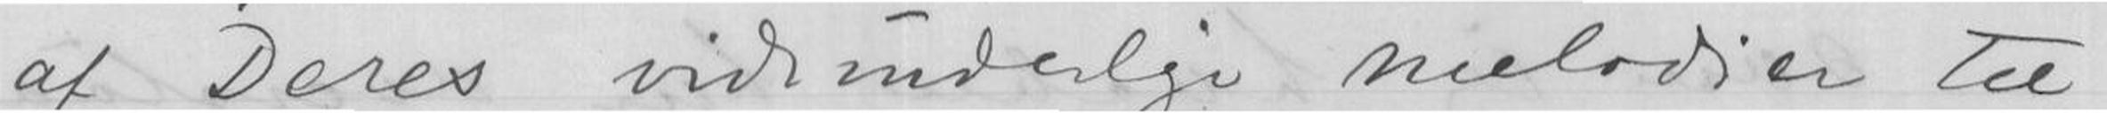af Deres vidunderlige melodier til
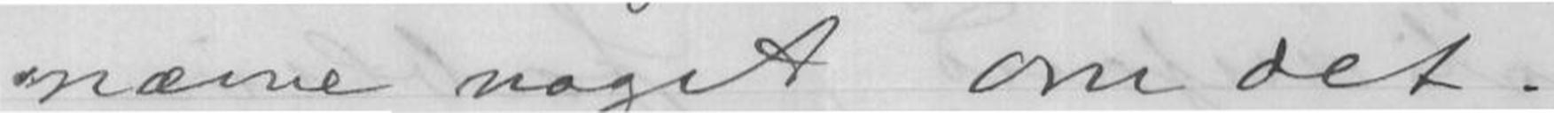nævne noget om det.
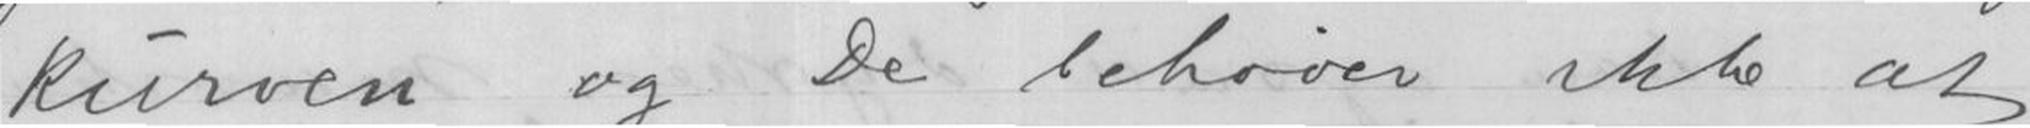kurven og De behøver ikke at
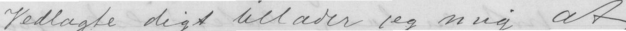Vedlagte digt tillader jeg mig at
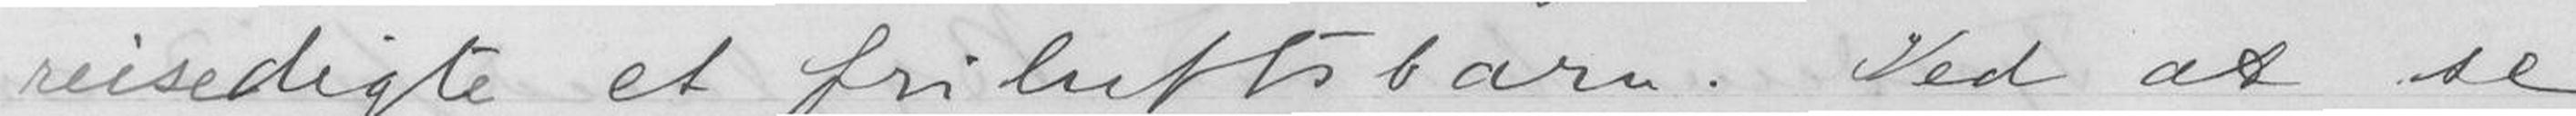reisedigt et friluftsbarn. Ved at se
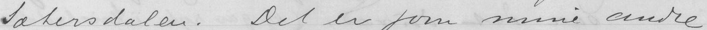Sælensdalen. Det er som mine andre
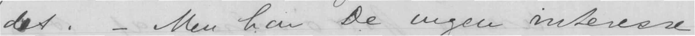det. - Men har De ingen interesse
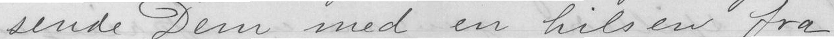sende Dem med en hilsen fra
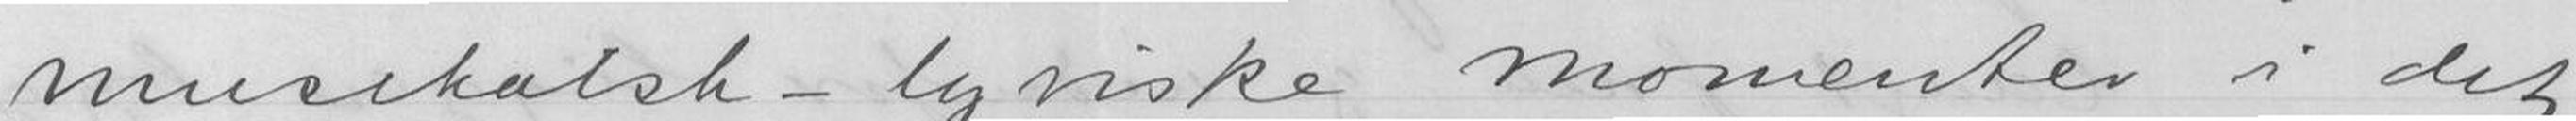musikalsk - lyriske momenter i det
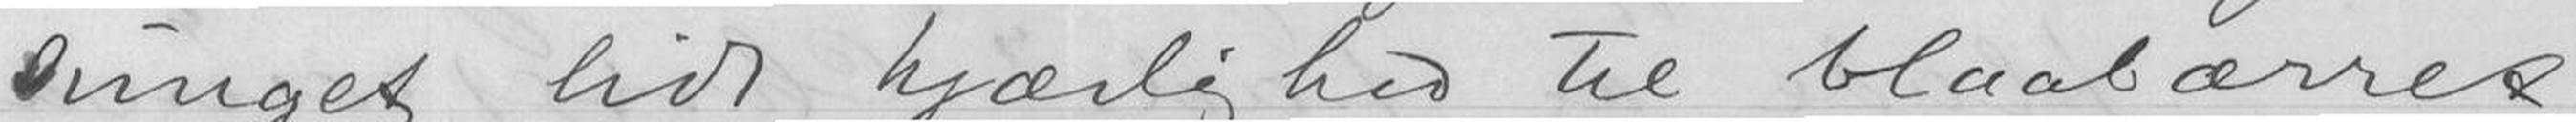svinget lidt kjærlighed til blaabærret
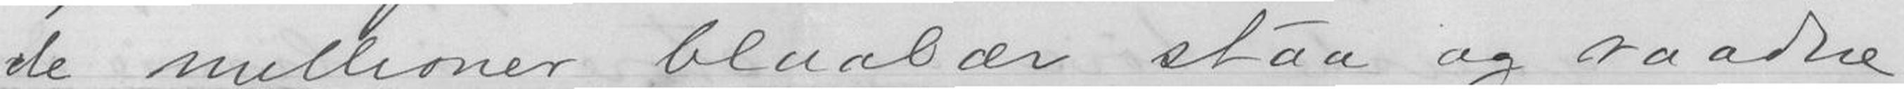de millioner blaabær staa og raadne
